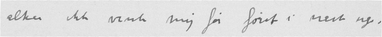altsaa ikke vente mig før først i neste uge.
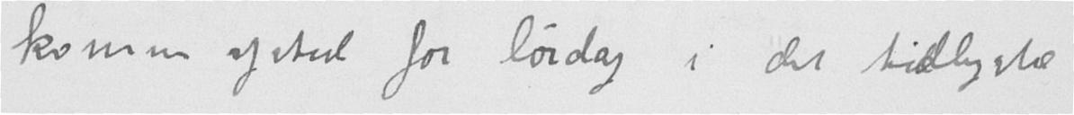komme afsted før lørdag i det tidligste
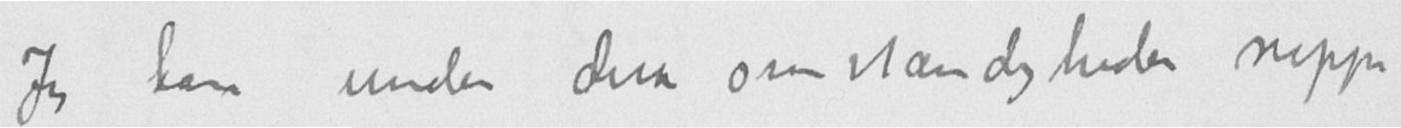Jeg kan under disse omstændigheder neppe
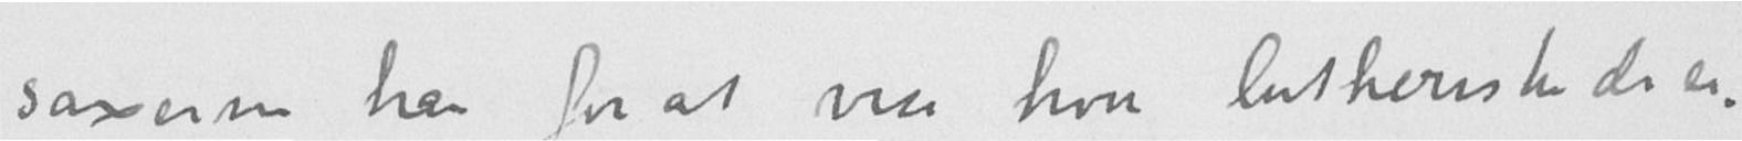saxerne har for at vise hvor lutherske de er.
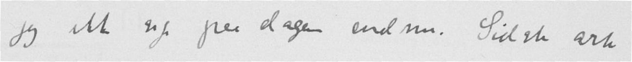jeg ikke sige paa dagen endnu. Sidste ark
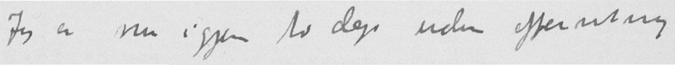Jeg er nu igjen to dager uden efterretning
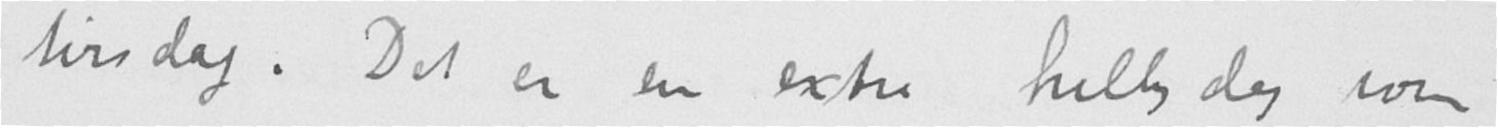tirsdag. Det er en extra helligdag som
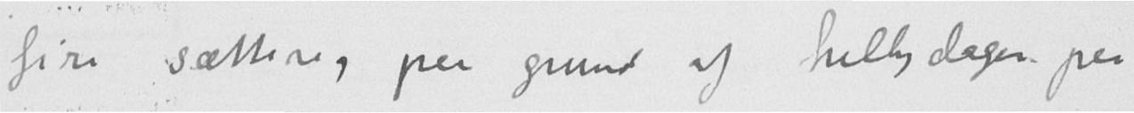fire sættere, paa grund af helligdagen paa
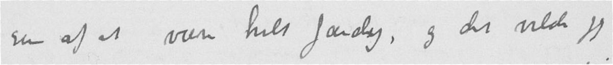sen af at være helt færdig, og det vilde jeg
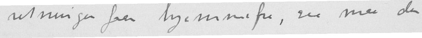retninger faar hjemmefra, saa maa du
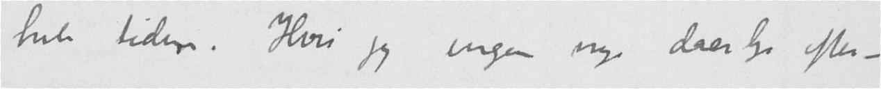hele tiden. Hvis jeg ingen nye daarlige efter-
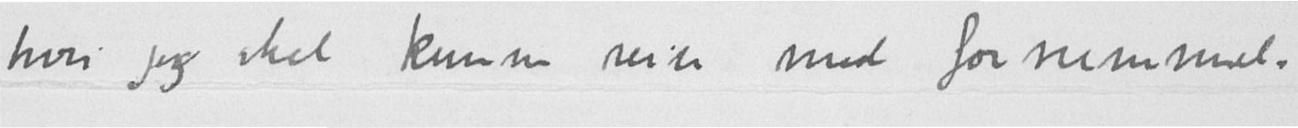hvis jeg skal kunne reise med fornemmel-
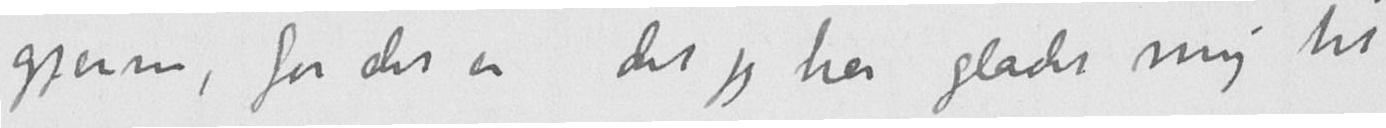gjerne, for det er det jeg har glædet mig til
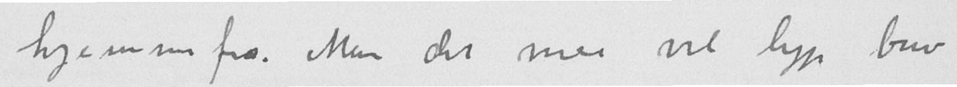hjemmefra. Men det maa vel ligge brev
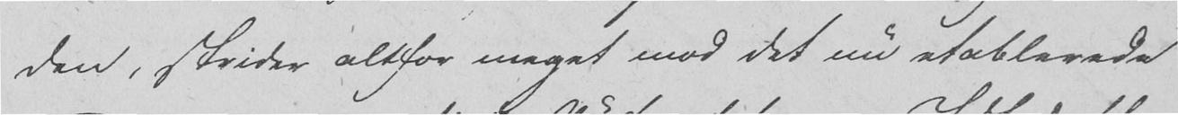den, strider altfor meget mod det nu etablerede
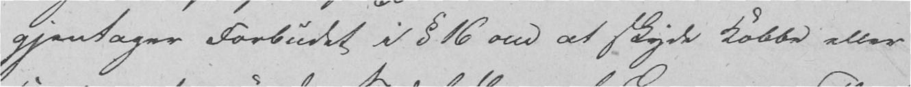gjentager Forbudet i § 16 om at skyde Kobbe eller
